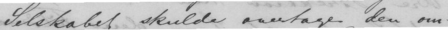Selskabet skulde overtage den om-
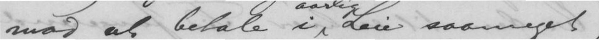mod at betale i Leie saameget,
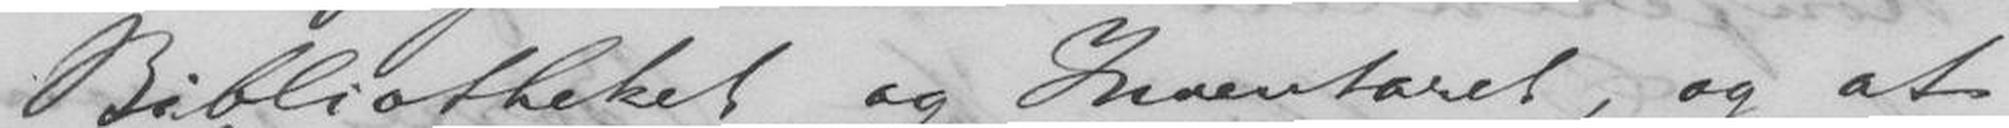Bibliotheket og Inventaret, og at
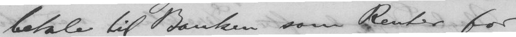betale til Banken som Renter for
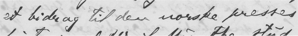et bidrag til den norske presses
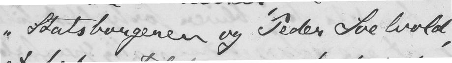"Statsborgeren og Peder Soelvold,
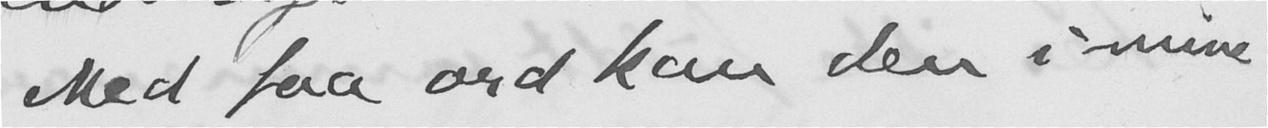Med faa ord kan den i mine
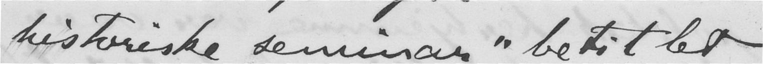historiske seminar", betitlet
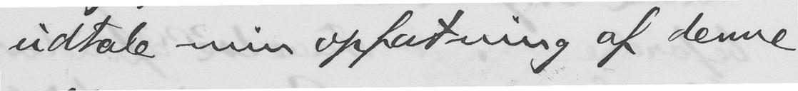udtale min opfatning af denne
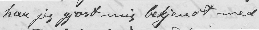har jeg gjort mig bekjendt med
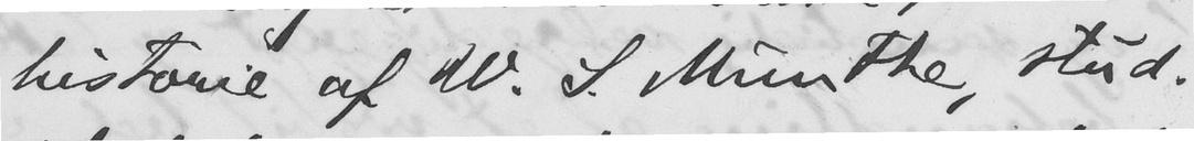historie af W. S. Munthe, stud.
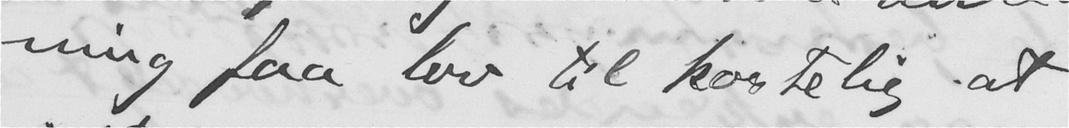ning faa lov til kortelig at
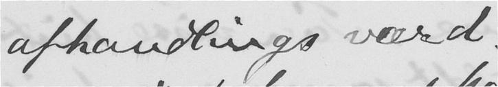afhandlings værd.
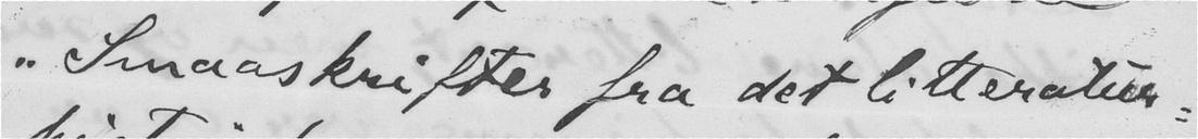"Smaaskrifter fra det litteratur-
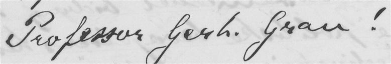Professor Gerh. Gran!
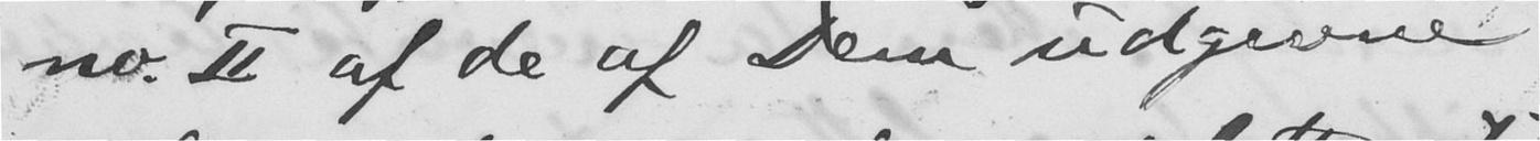no II af de af Dem udgivne
